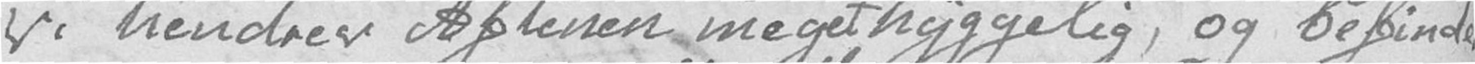Vi hendrev Aftenen meget hyggelig, og befinder
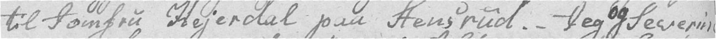til Jomfru Hejerdal paa Stensrud. – Jeg og Severine
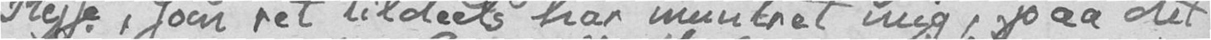Rejse, som ret tildeels har muntret mig, paa det
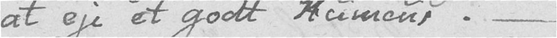at eje et godt Humeur. –
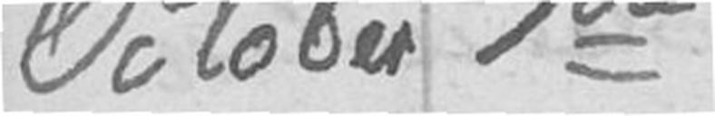October 7de
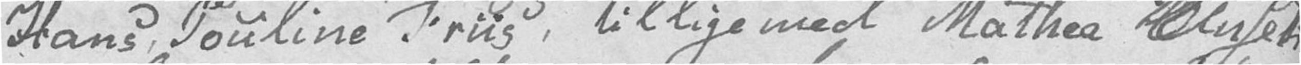Hans, Pouline Friis, tillige med Mathea Olnset.
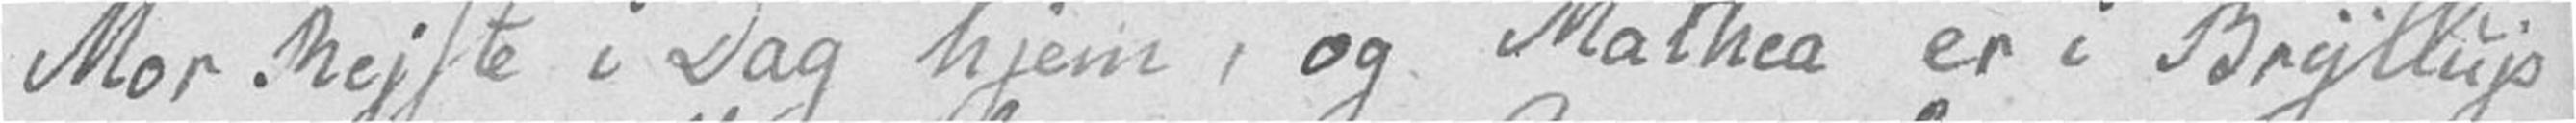Mor Rejste i Dag hjem, og Mathea er i Bryllup
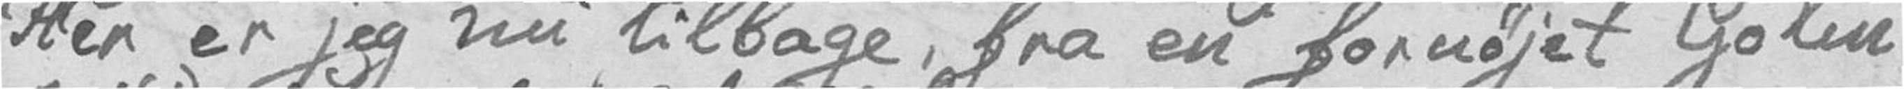Her er jeg nu tilbage, fra en fornøjet Golin
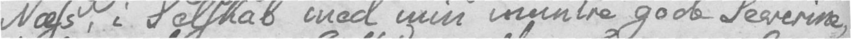Næss, i Selskab med min muntre gode Severine,
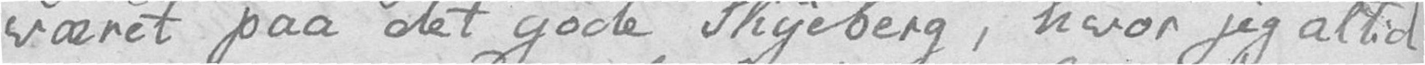været paa det gode Skyeberg, hvor jeg altid
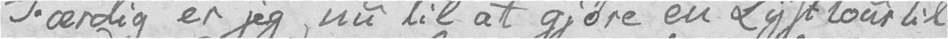Færdig er jeg nu til at gjøre en Lysttour til
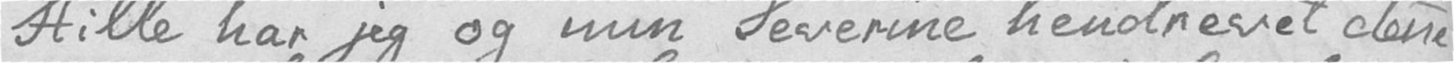Stille har jeg og min Severine hendrevet denne
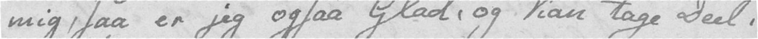mig, saa er jeg ogsaa Glad, og kan tage Deel i
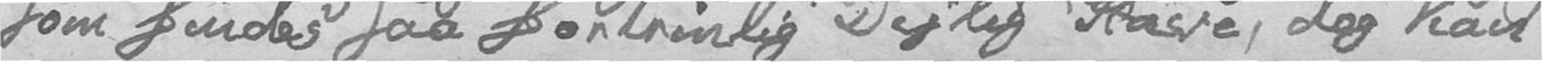som findes saa fortrinlig Dejlig Have, dog kan
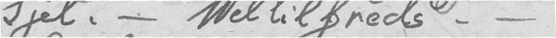Sjel. – Veltilfreds. –
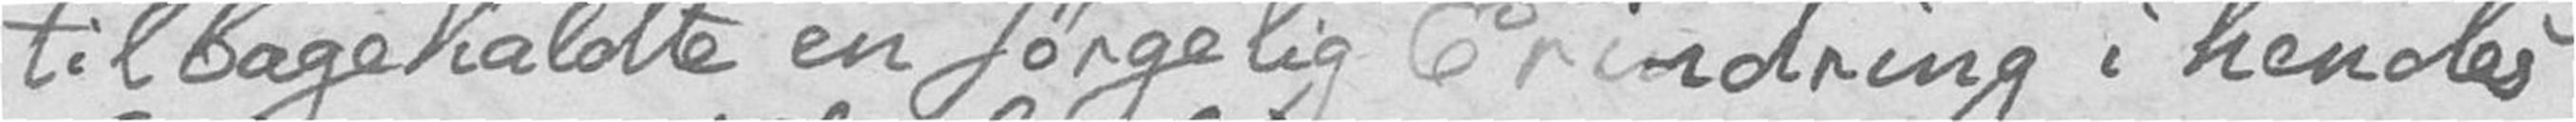tilbagekaldte en sørgelig Erindring i hendes
